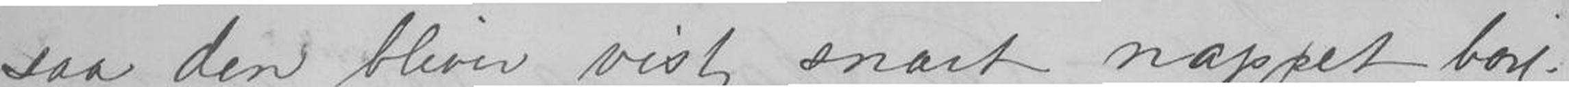saa den bliver vist snart nappet bort.
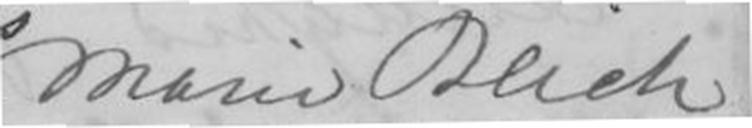Marie Blich
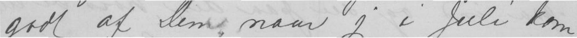godt af Dem, naar jeg i Juli kom-
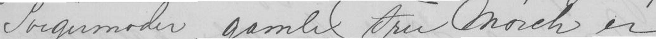Svigermoder, gamle Fru Mørch er
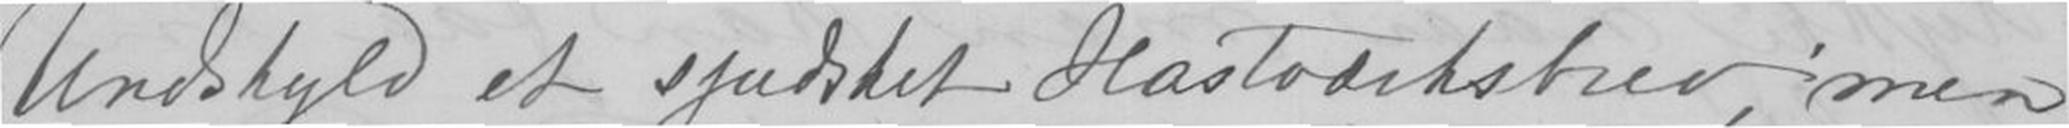Undskyld et sjudsket Hastværksbrev, men
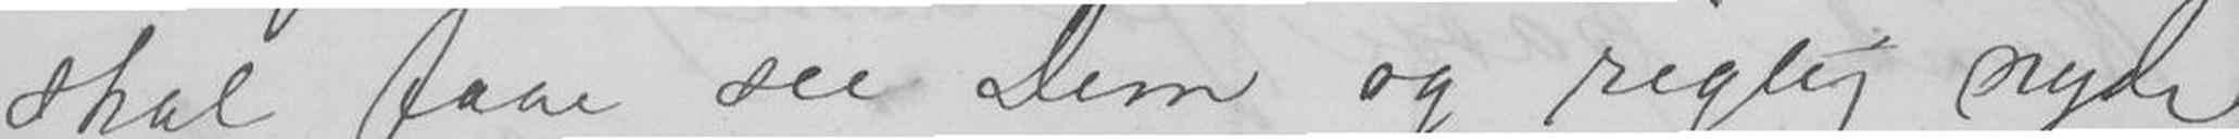skal faae see Dem og rigtig nyde
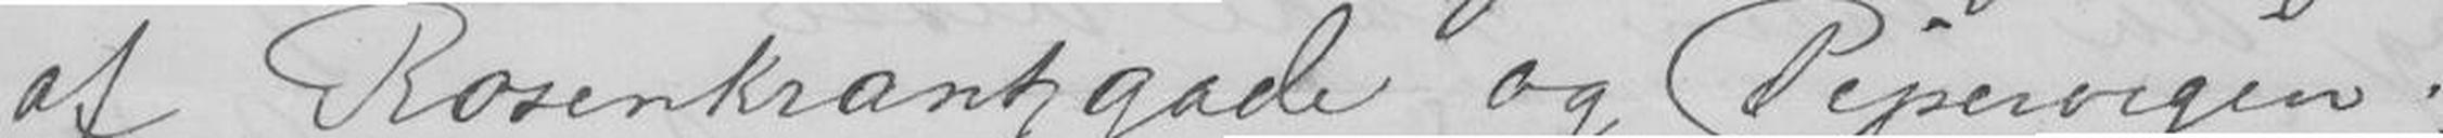af Rosenkranzgade og Pipervigen;
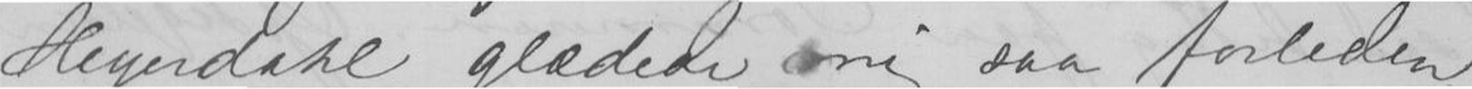Heyerdahl glædede mig saa forleden
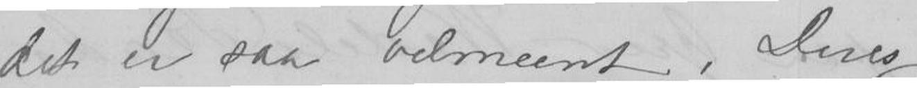det er saa velment. Deres
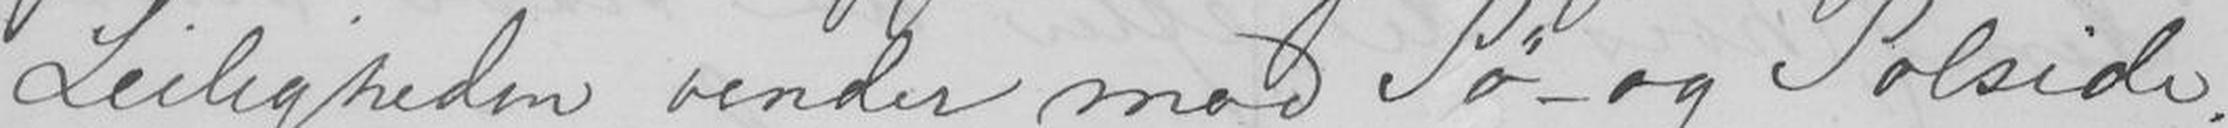Leiligheden vender mod Sø- og Solside.
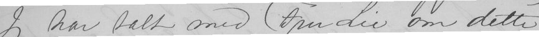Jeg har talt med Fru Lie om dette,
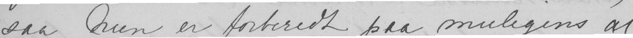saa hun er forberedt paa muligens at
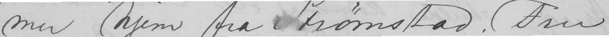mer hjem fra Strømstad. Fru
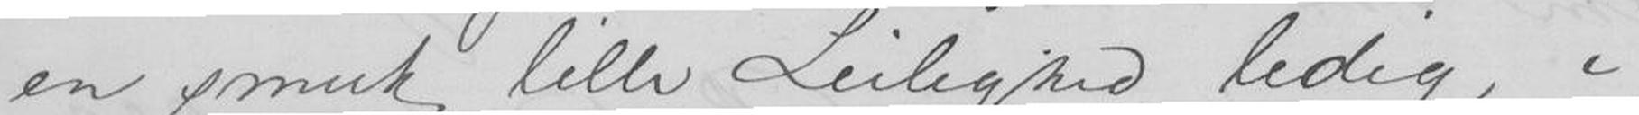en smuk lille Leilighed ledig i
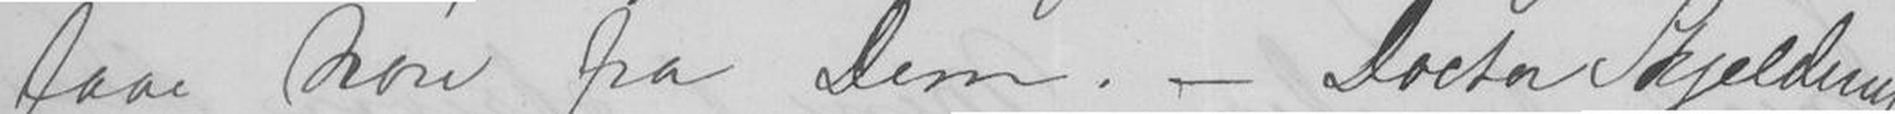faae høre fra Dem. - Doctor Skjelderups
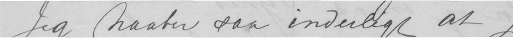-Jeg haaber saa inderligt at jeg
